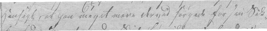Hensigt, at han meget mere derved sørgede for sin Sik-
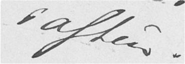i aften.
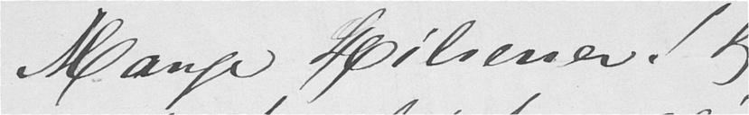Mange Hilsener! Jeg
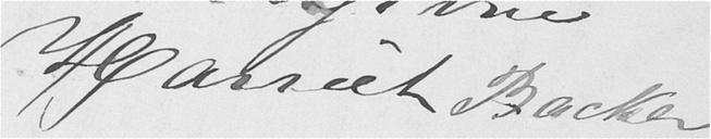Harriet Backer
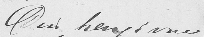Din hengivne
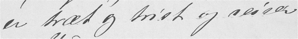er træt og trist og reiser
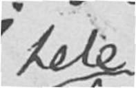hele
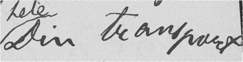Din transport,
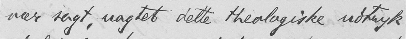nær sagt, uagtet dette theologiske udtryk
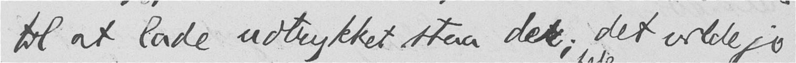til at lade udtrykket staa der; det, vilde jo
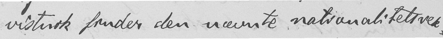vistnok finder den nævnte nationalitetsvek-
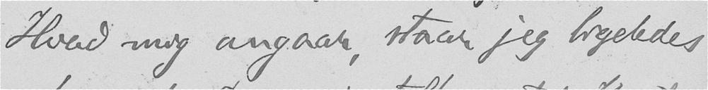Hvad mig angaar, staar jeg ligeledes
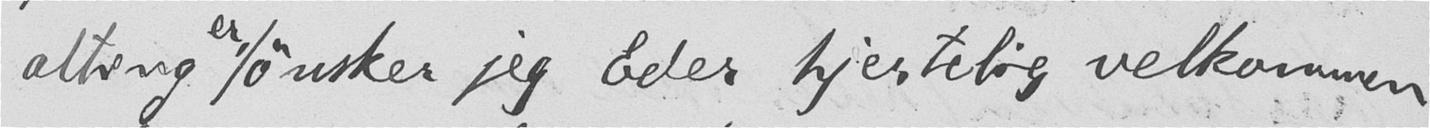alting er ønsker jeg Eder hjertelig velkommen,
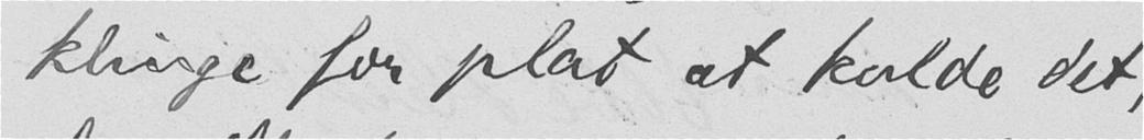klinge for plat at kalde det
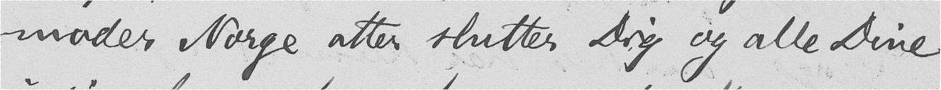moder Norge atter slutter Dig og alle Dine
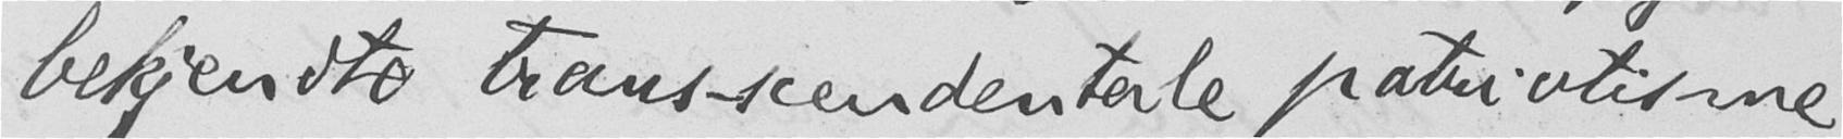bekjendte trans-scendentale patriatisme
